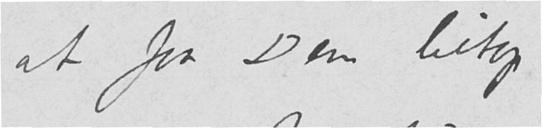at faa Dem hitop.
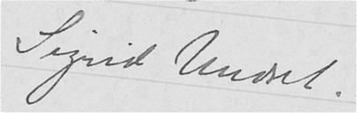Sigrid Undset.
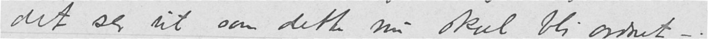det ser ut som dette nu skal bli ordnet –.
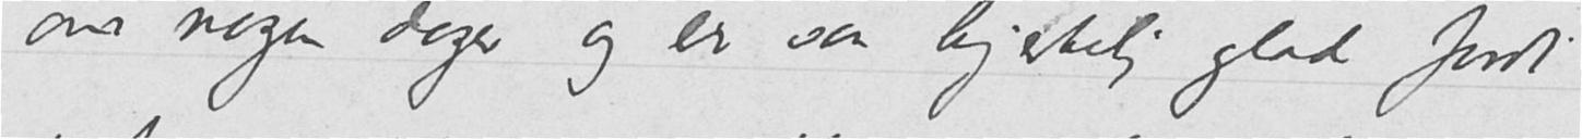om nogen dager og er saa hjertelig glad fordi
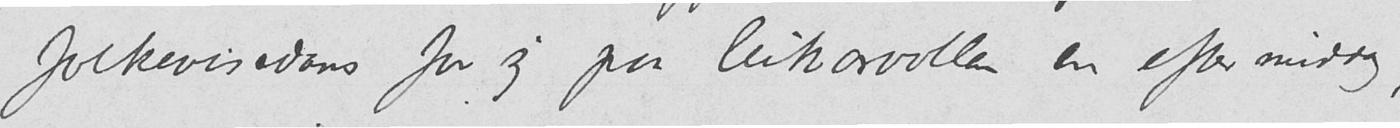folkevisedans for sig paa leikarvollen en eftermiddag,
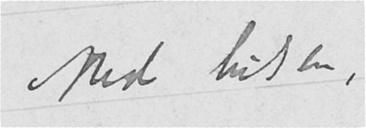Med hilsen,
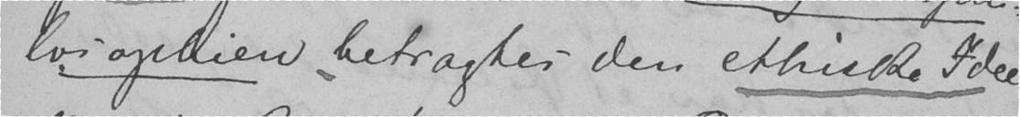losophien betragtes den ethiske Idee
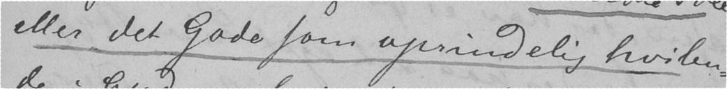eller det Gode som oprindelig hvilen-
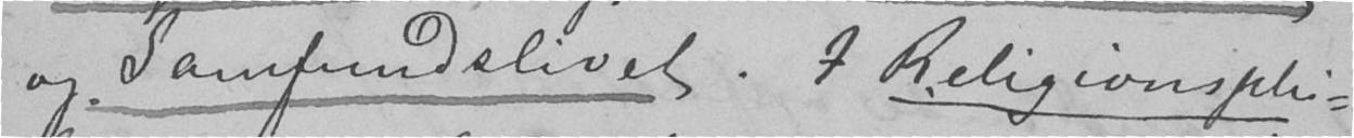og Samfundslivet. I Religionsphi-
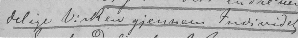delige Virken gjennem Individet
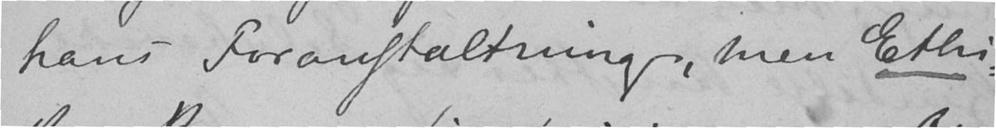hans Foranstaltning, men Ethi-
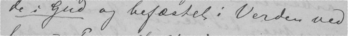de i Gud og befæstet i Verden ved
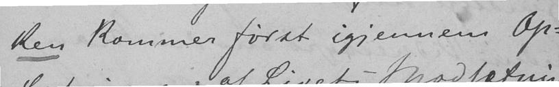ken kommer først igjennem Op-
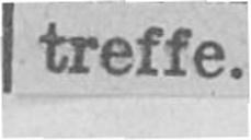treffe.
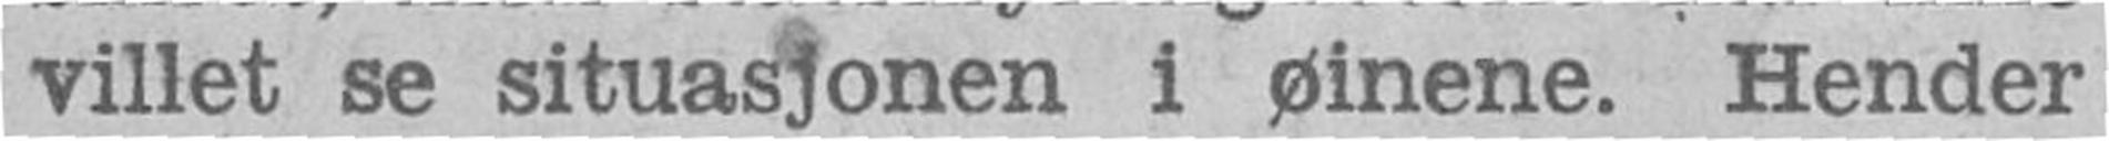villet se situasjonen i øinene. Hender
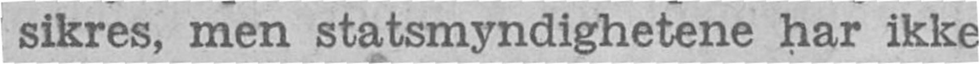sikres, men statsmyndighetene har ikke
